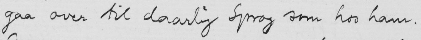gaa over til daarlig Sprog som hos ham.
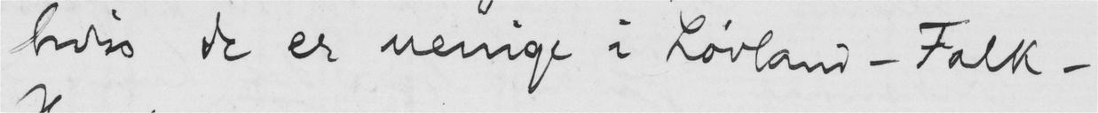hvis de er uenige i Løvland - Falk -
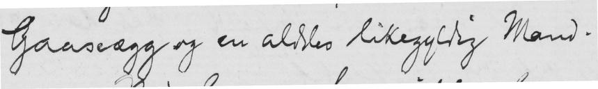Gaaseægg og en aldeles likegyldig Mand.
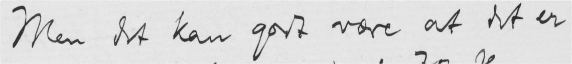Men det kan godt være at det er
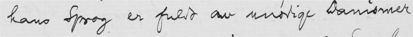hans Sprog er fuld av unødige Danismer
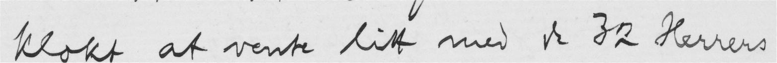klokt at vente litt med de 32 Herrers
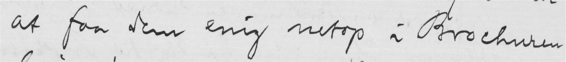at faa dem enig netop i Brochuren
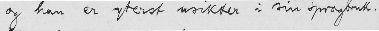og han er yterst usikker i sin Sprogbruk.
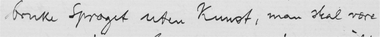bruke Sproget uten Kunst, man skal være
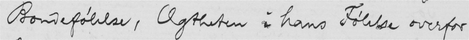Bondefølelse, Ægtheten i hans Følelse overfor
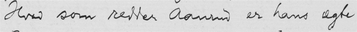Hvad som redder Aanrud er hans ægte
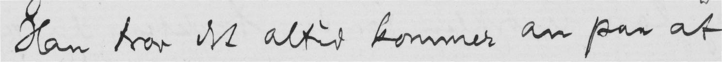Han tror det altid kommer an paa at
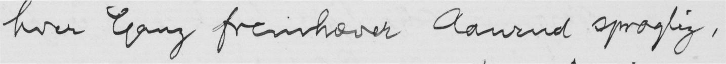hver Gang fremhæver Aanrud sproglig,
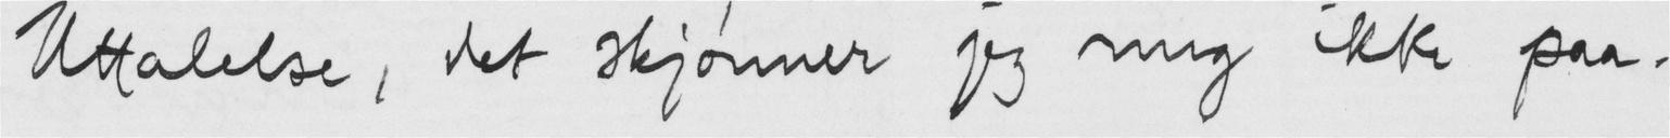Uttalelse, det skjønner jeg mig ikke paa.
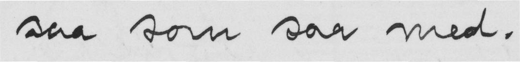saa som saa med.
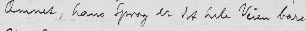Æmnet, hans Sprog er det hele Veien bare
